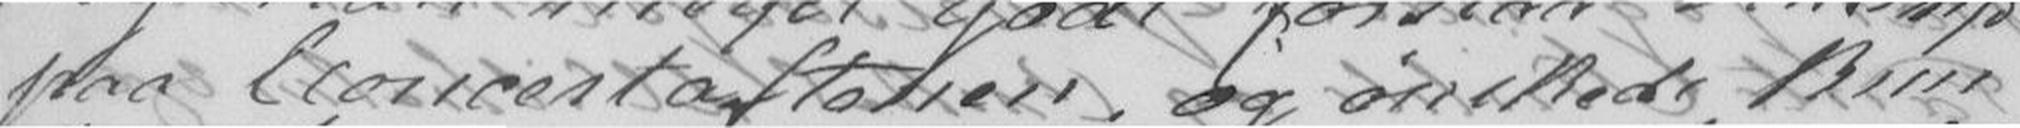paa Concertaftenen, og ønskede kun
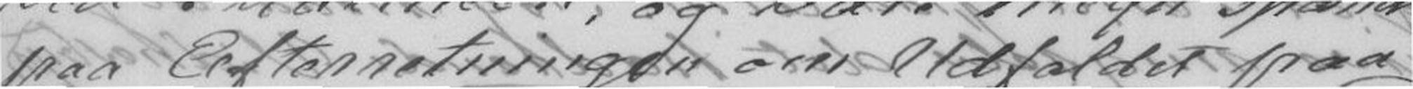paa Efterretningen om Udfaldet paa
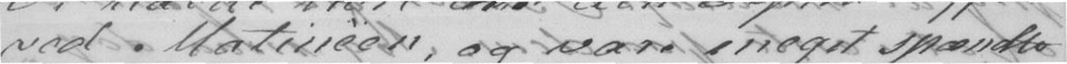ret Matineer, og vare meget spændte
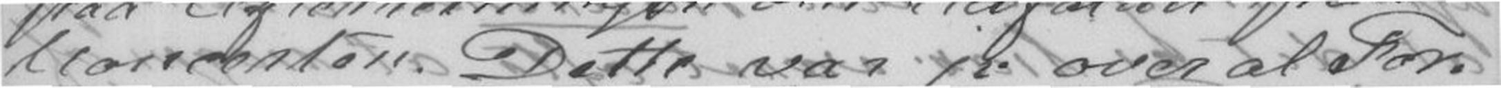Concerten. Dette var jo over al For-
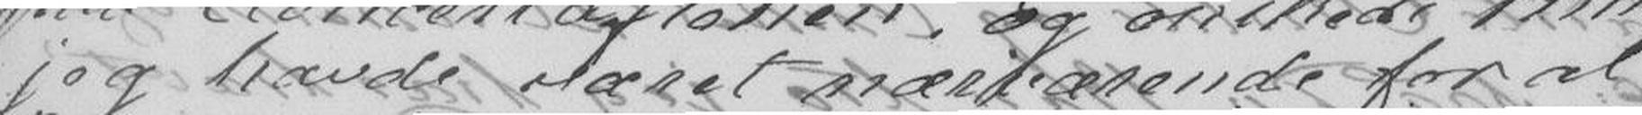jeg havde været nærværende for at
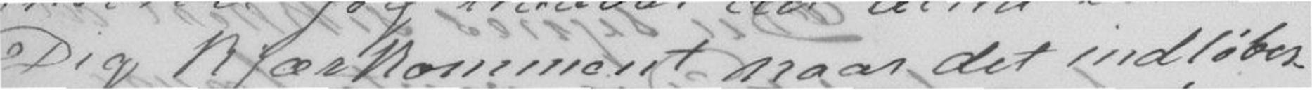Dig kjærkomment naar det indløbes.
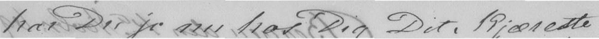har Du jo nu hos Dig Dit, Kjæreste
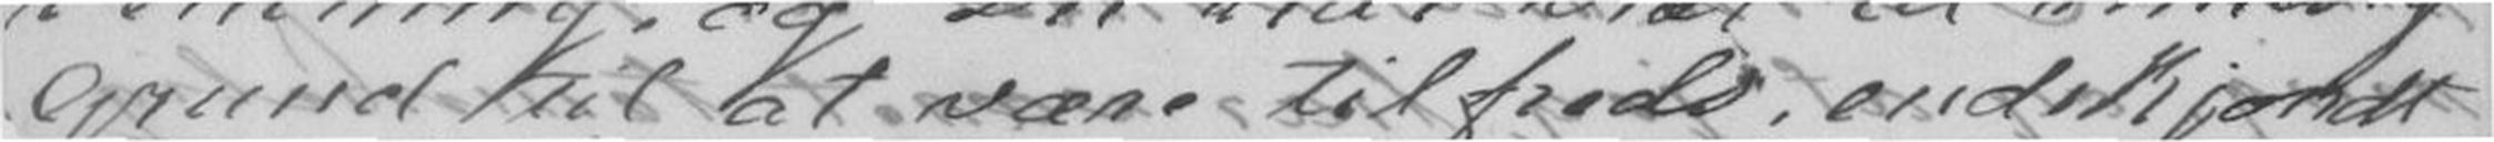Grund til at være til freds, endskjøndt
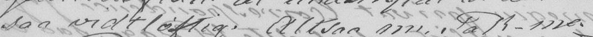saa vidtløftig. Altsaa nu, Tak - me-
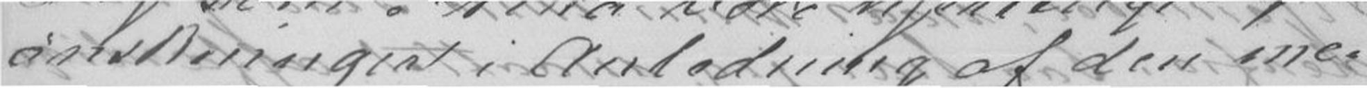ønskninger i Anledning af den me-
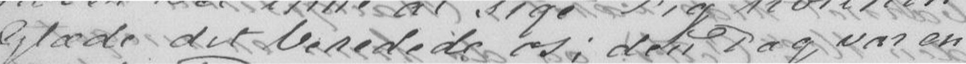Glæde det beredede os; den Dag var en
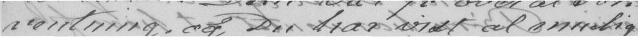ventning, og Du har vist al muelig
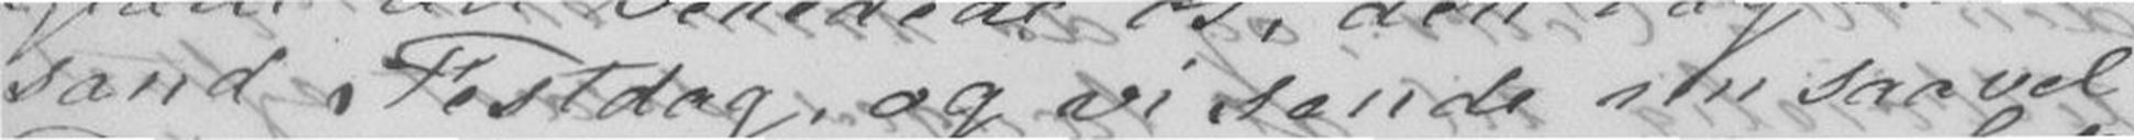sand Festdag, og vi sende nu saavel
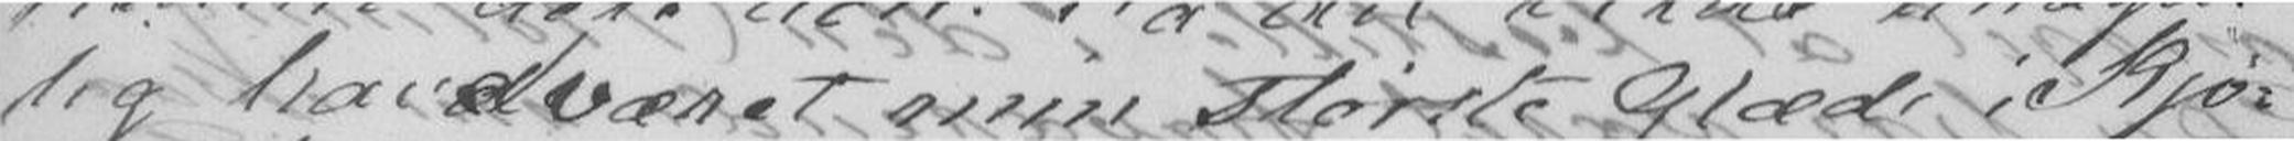lighavd været min største Glæde, Kjø-
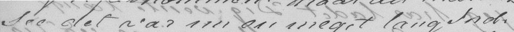See det var nu en megen lang Ind-
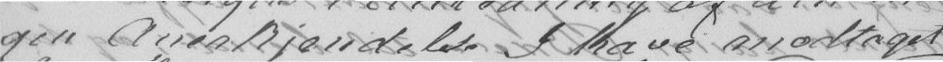gen Anerkjendelse I have modtaget.
